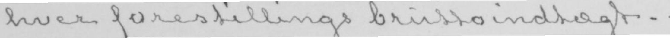hver forestillings bruttoindtægt.-
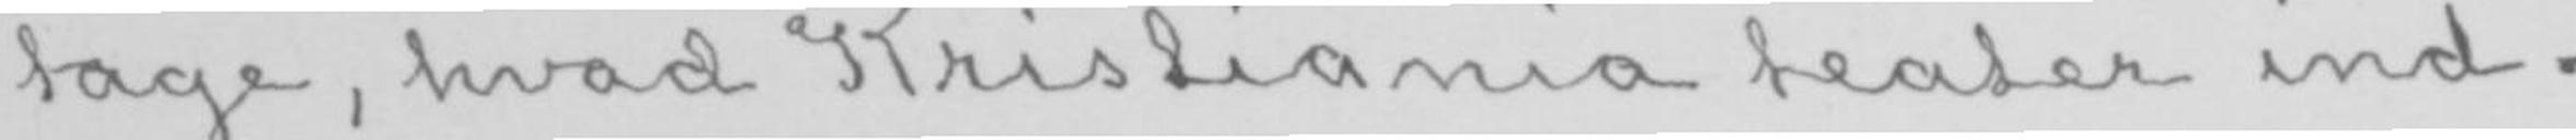tage, hvad Kristiania teater ind-
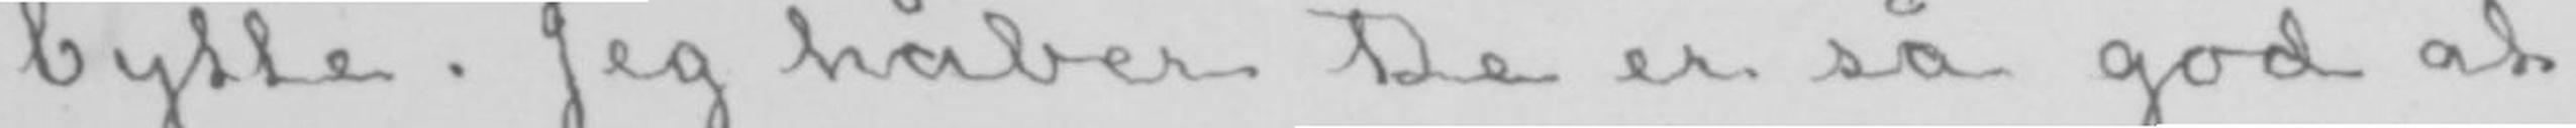bytte. Jeg håber De er så god at
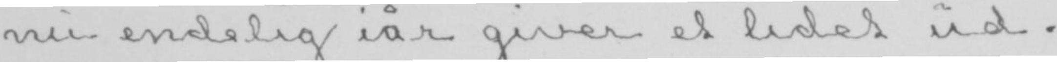nu endelig iår giver et lidet ud-
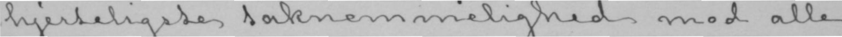hjerteligste taknemmelighed mod alle
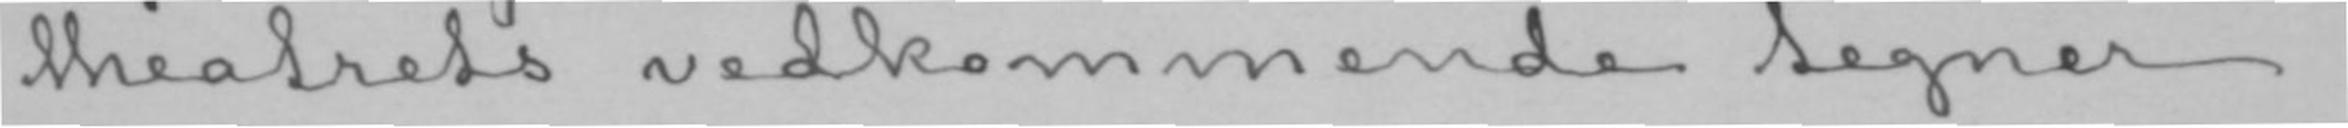theatrets vedkommende tegner jeg
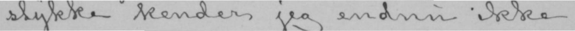stykke kender jeg endnu ikke.
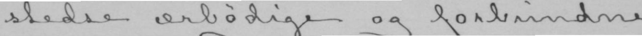stedse ærbødige og forbundne
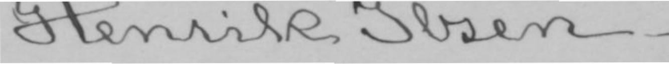Henrik Ibsen.
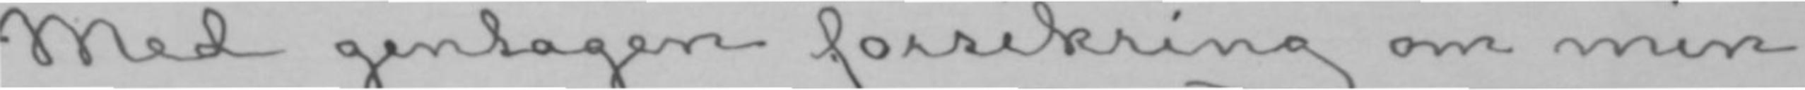Med gentagen forsikring om min
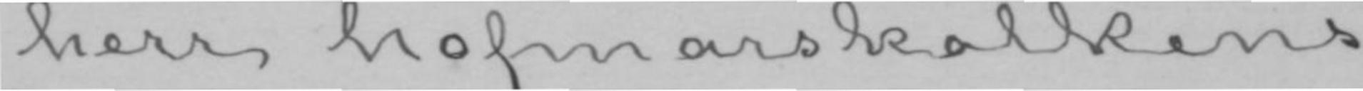herr hofmarskalkens
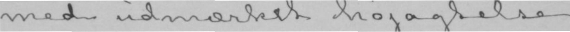med udmærket højagtelse
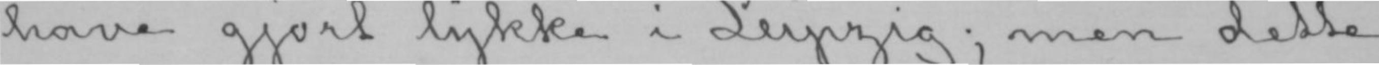have gjort lykke i Leipzig; men dette
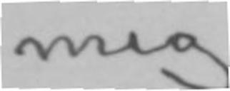mig
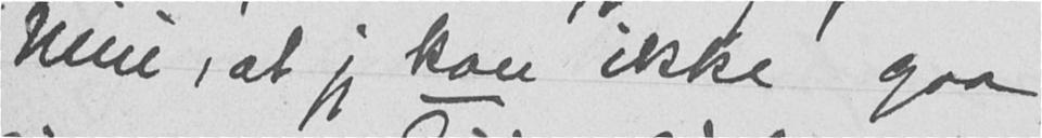Nini, at jeg kan ikke gaa
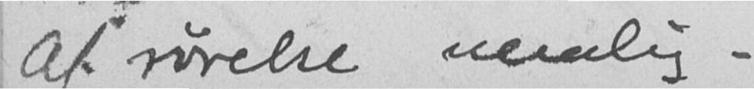af rørelse nemlig.
